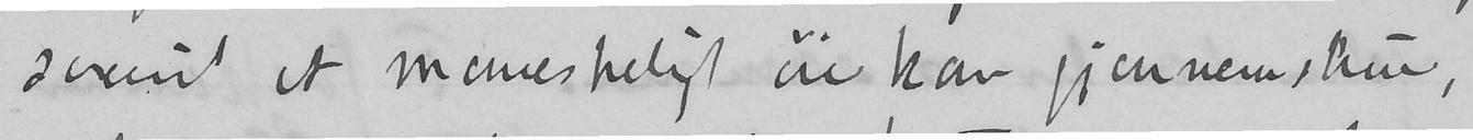saavidt et menneskeligt øie kan gjennemskue,
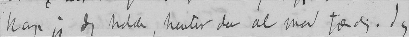kan jeg dog holde, henter da al mad færdig. Jeg
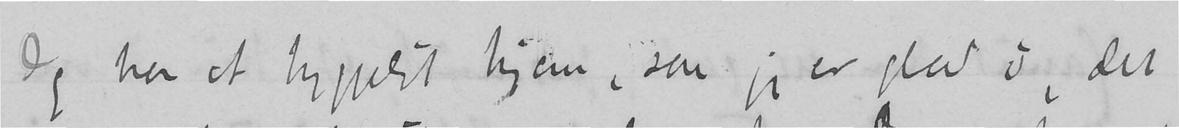Jeg har et hyggeligt hjem, som jeg er glad i, det
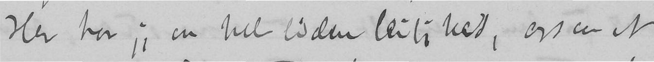Her har jeg en hel liden leilighed, ogsaa et
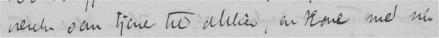værelse som tjener til atelier, en kone med sin
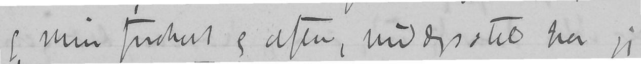og min frokost og aften, middagsstel har jeg
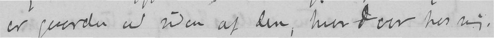er gaarden ved siden af den, hvor I var hos mig.
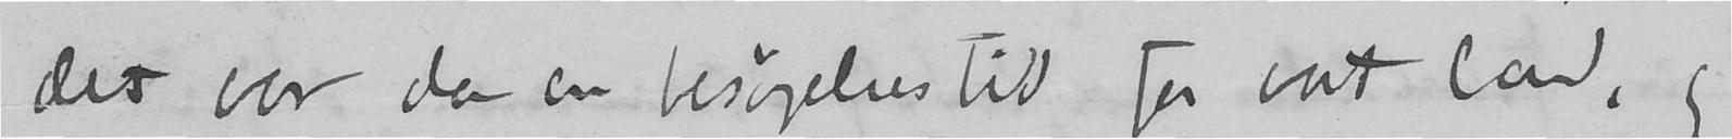det var da en besøgelsestid for vort land, og
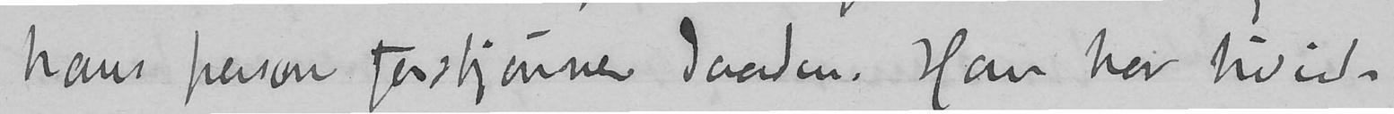hans person forskjønner daaden. Han har hidind-
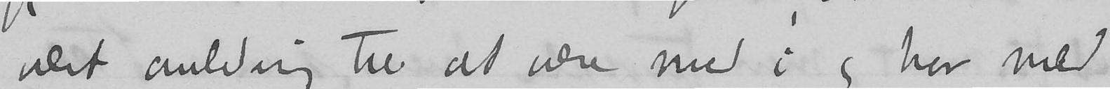vært anledning til at være med i og har med
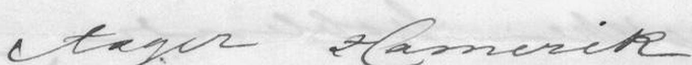Asger Hamerik
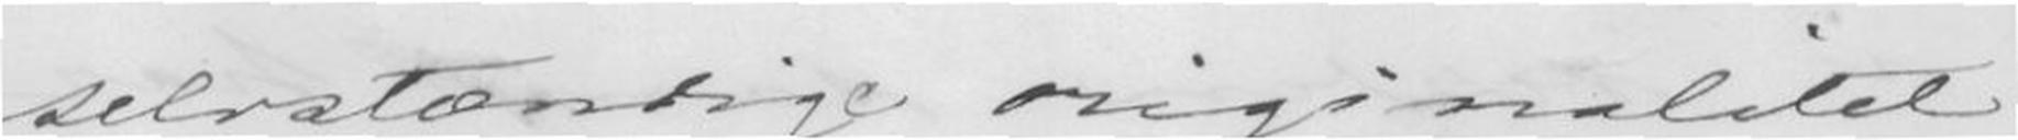selvstændige originalitet
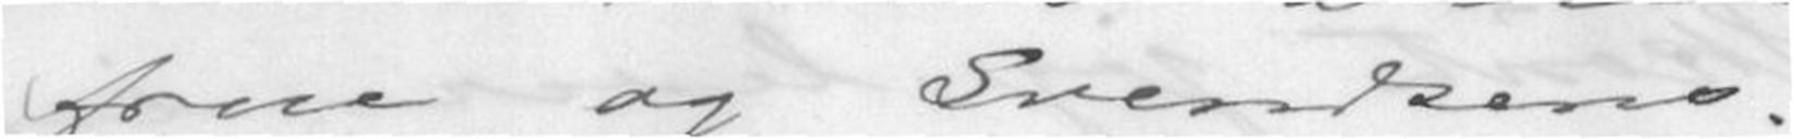frue og Svendsen
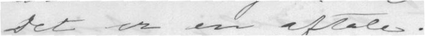Det er en avtale.
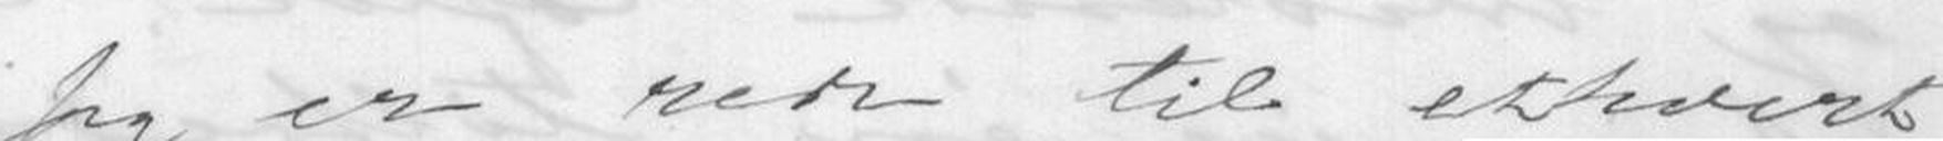Jeg er rede til ethvert
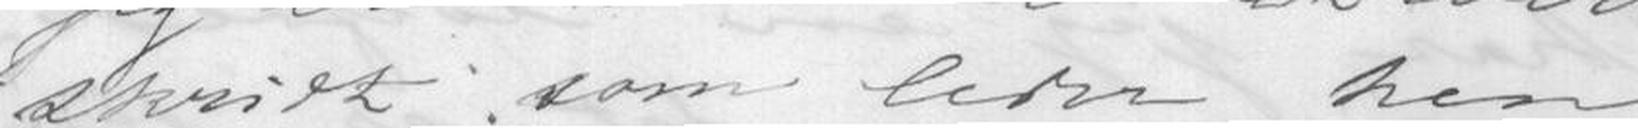skridt som leder hen
